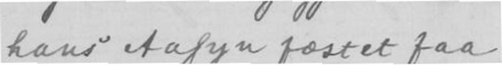hans Aasyn fæstet faa,
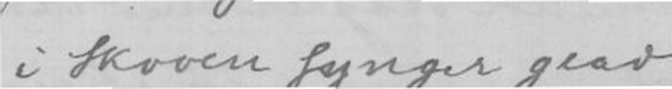i Skoven synger glad
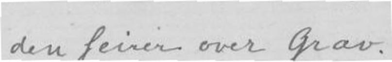den feirer over Grav.
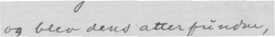og blev dens atterfunden,
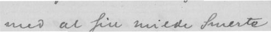med al sin milde Smerte
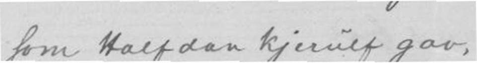som Halfdan Kjerulf gav,
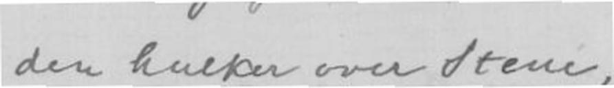den hulker over Stene,
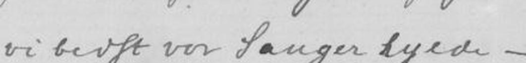vi bedst vor Sangen hylde -
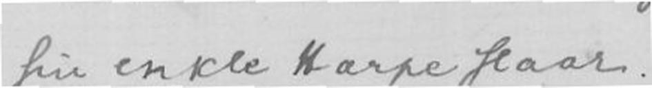sin enkle Harpe slaar.
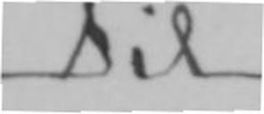Til
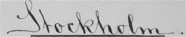Stockholm.
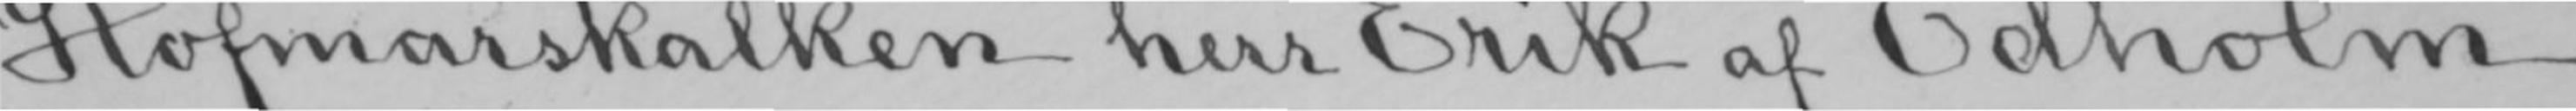Hofmarskalken herr Erik af Edholm.
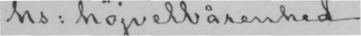hs: højvelbårenhed
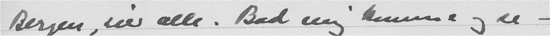Bergen, sier alle. Bad mig komme og se -
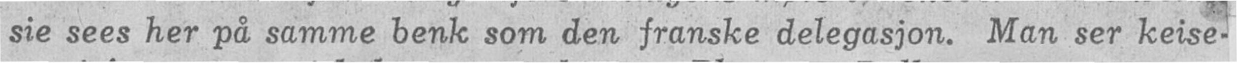sie sees her på samme benk som den franske delegasjon. Man ser keise-
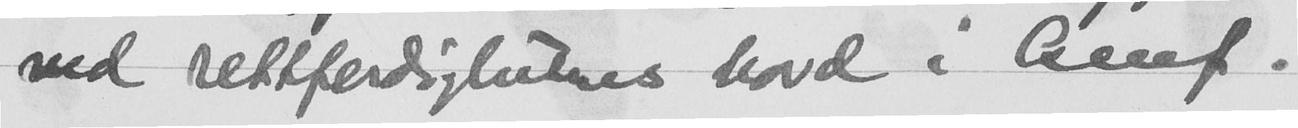ved rettferdighetens bord i Amf.
